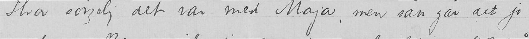Hvor sørgelig det var med Maja, men san gar det jo
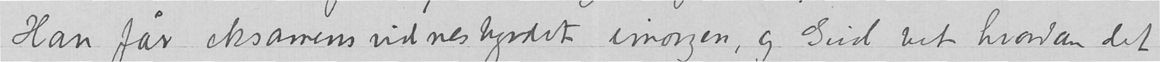Han far eksamens vidnestyrdet imorgen, og Gud vet hvordan det
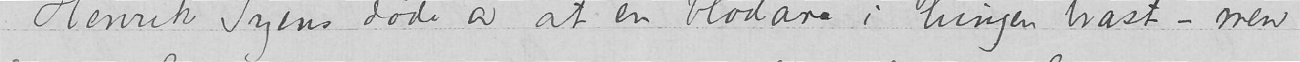Henrik Irgens døde av at en blodåre i bringen brast – men
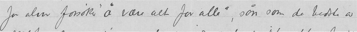for alvor forsøke å være alt for alle, søn som de bedste av
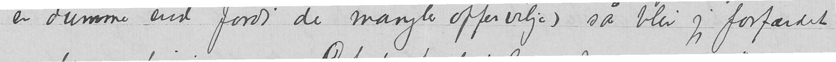er dumme end fordi de mangler offervilje) sa blir jeg forfærdet
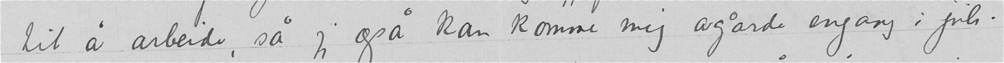til av arbeide, så jeg ogsa kan komme mig agarde engang i juli.
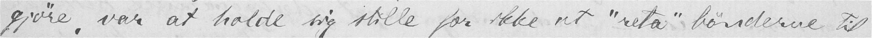gjøre, var at holde sig stille for ikke at "reta" bønderne til
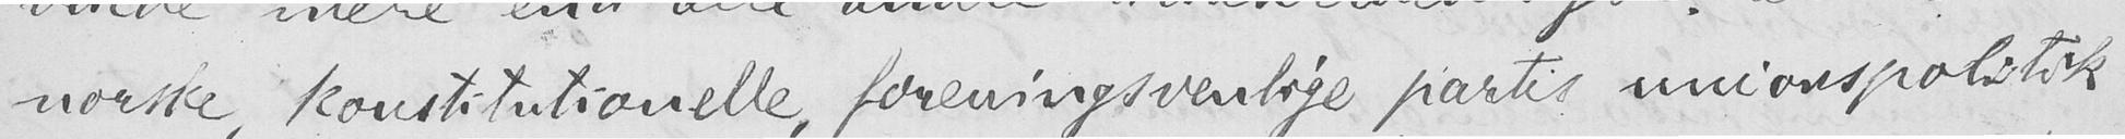norske, konstitutionelle, foreningsvenlige partis unionspolitik
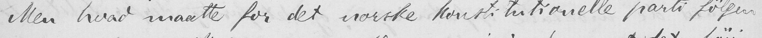Men hvad maatte for det norske konstitutionelle parti følgen
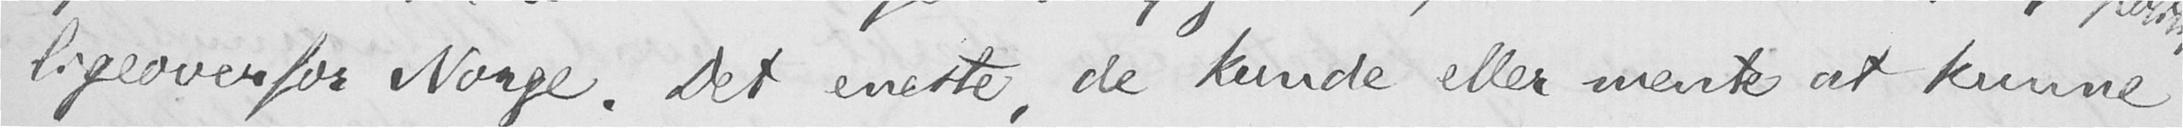ligeoverfor Norge. Det eneste, de kunde eller mente at kunne
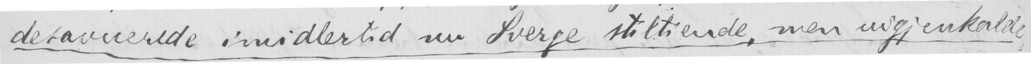desavuerede imidlertid nu Sverge stiltiende, men uigjenkalde-
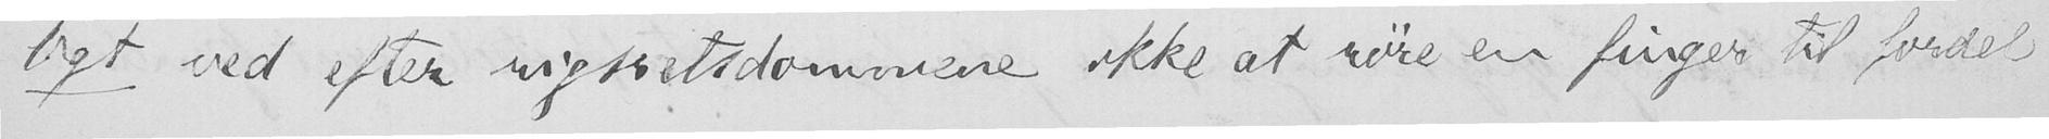ligt ved efter rigsretsdommene ikke at røre en finger til fordel
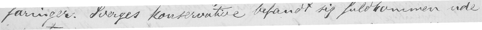faringer. Sverges konservative befandt sig fuldkommen ude
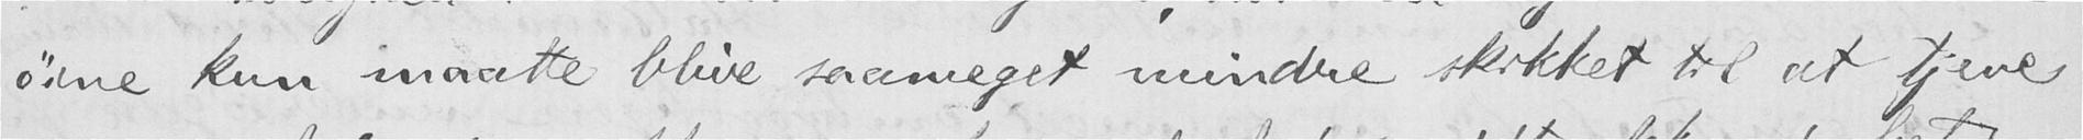øine kun maatte blive saameget mindre skikket til at tjene
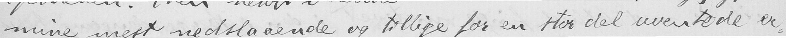mine mest nedslaaende og tillige for en stor del uventede er-
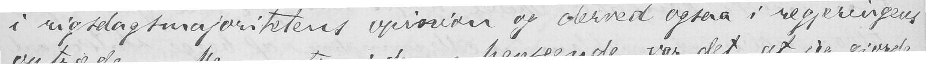i rigsdagsmajoritetens opinion og derved ogsaa i regjeringens
